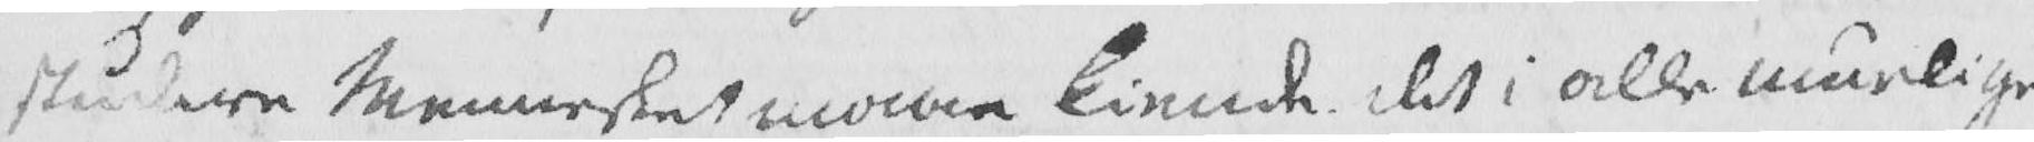studere Mennesket maae kiende det i alle muelige
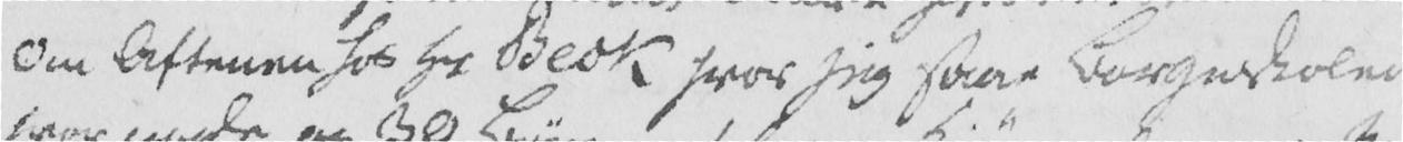Om Aftenen hos Hr Beck hvor jeg saae Borgerskolen
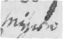Mission
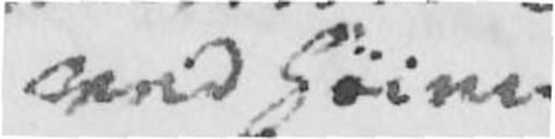med Høien
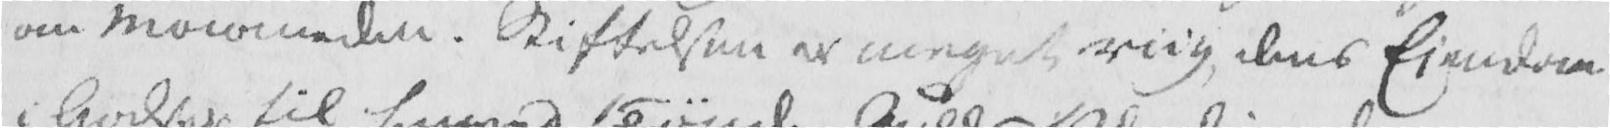om Maaneden. Stiftelsen er meget riig, dens Eiendom
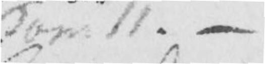Tom II. -
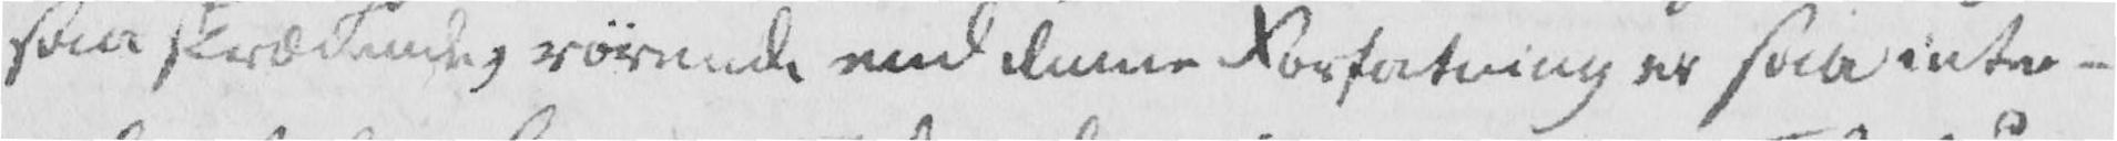saa skræckelig rørende end denne Fortatning er saa inte-
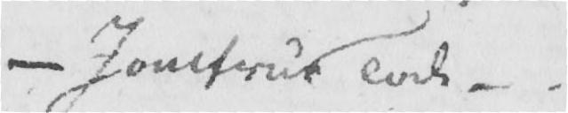- Jomfrue end - .
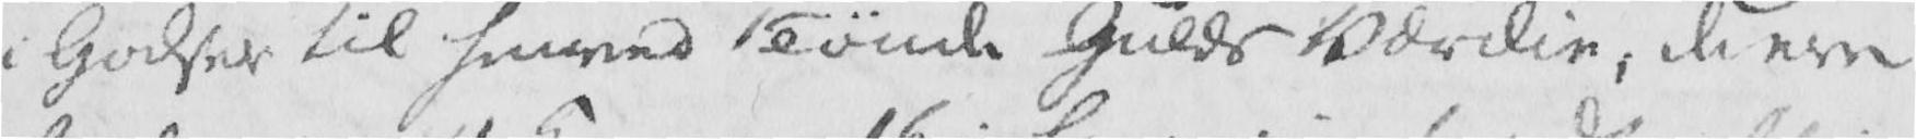i Godser til henved 1 Tønde Gulds Værdie, de ere
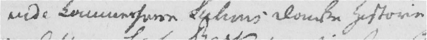undi Kammerherre Suhms danske Historie
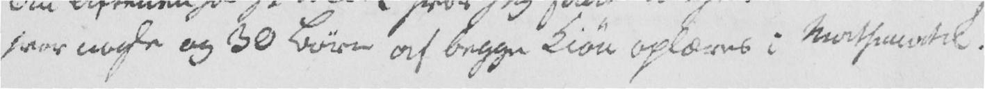hvor nogle og 30 Børn af begge Kiøn oplæres i Mathematik.
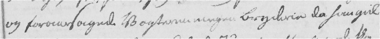og foraarsagede Vogterne megen Bryderie da han gik
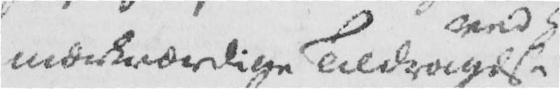mærkværdige Tildragelse paa Lækol i Annnedallen
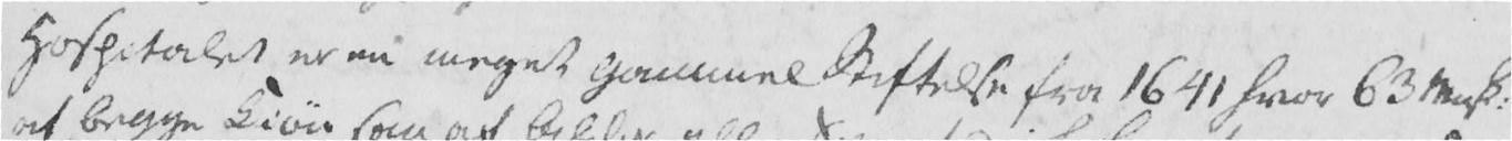Hospitalet er en meget gammel Stiftelse fra 1641 hvor 63 Mnsk.
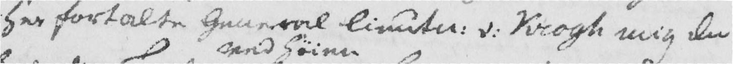Her fortalte General lieutn. v. Krogh mig den
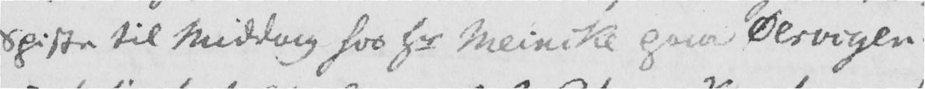Spiste til Middag hos Hr Meincke paa Ølsvigen.
